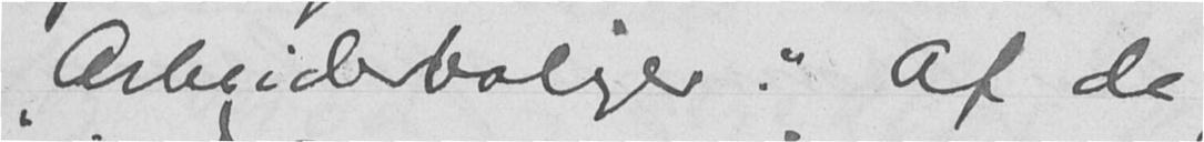"arbeiderboliger." Af de
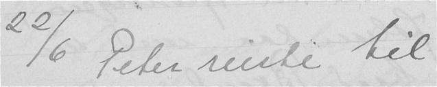22/6 Peter reiste til
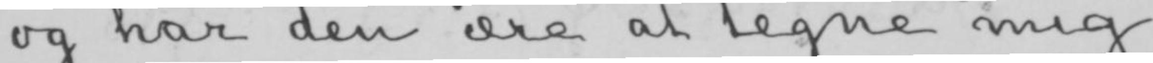og har den ære at tegne mig
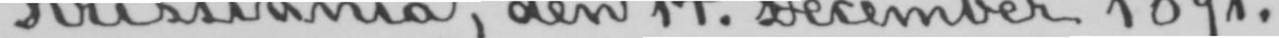Kristiania, den 14. December 1891.
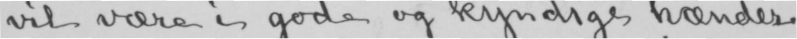vil være i gode og kyndige hænder.
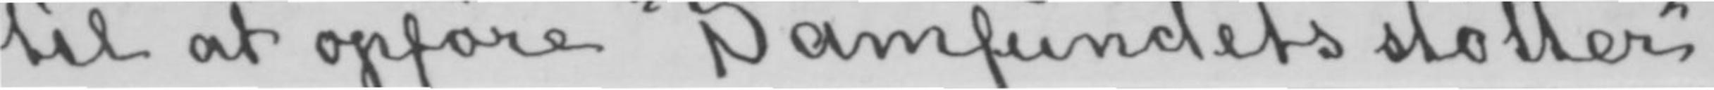til at opføre «Samfundets støtter»
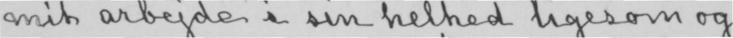mit arbejde i sin helhed ligesom og-
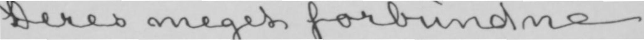Deres meget forbundne
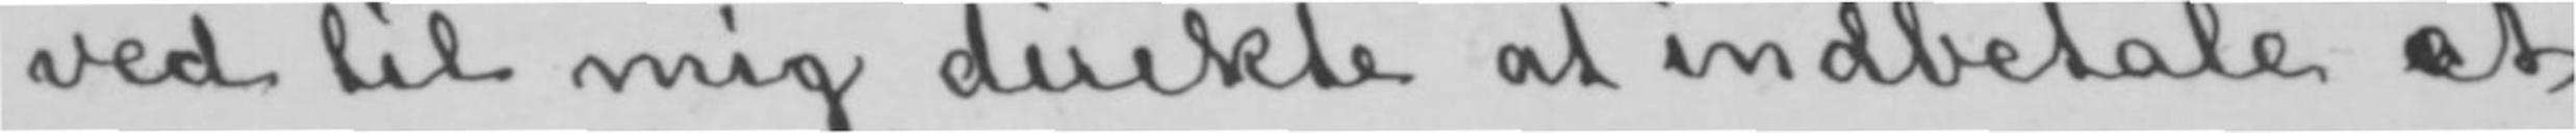ved til mig direkte at indbetale et
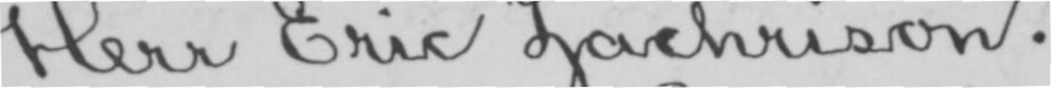Herr Eric Zachrison.
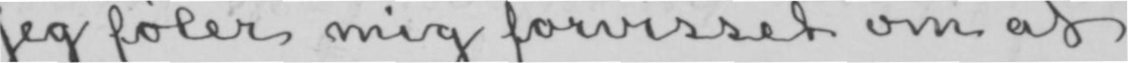Jeg føler mig forvisset om at
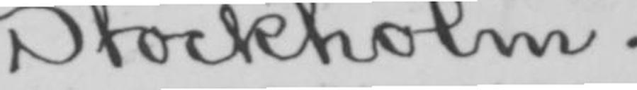Stockholm.
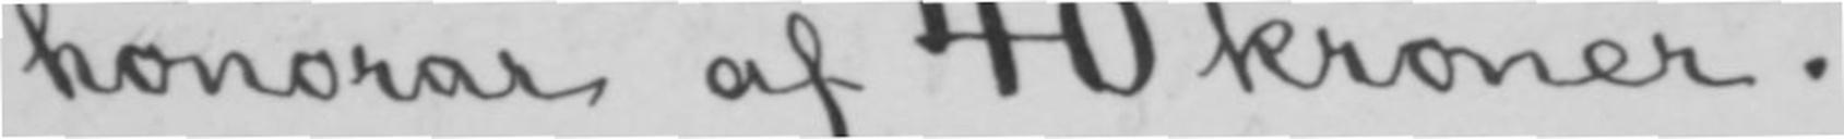honorar af 40 kroner.
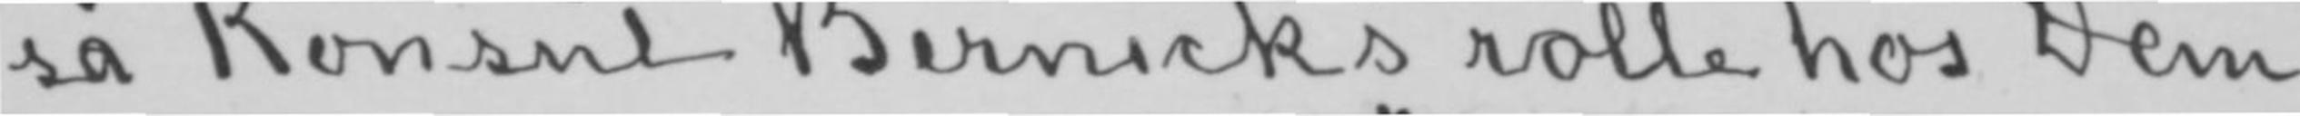så Konsul Bernick's rolle hos Dem
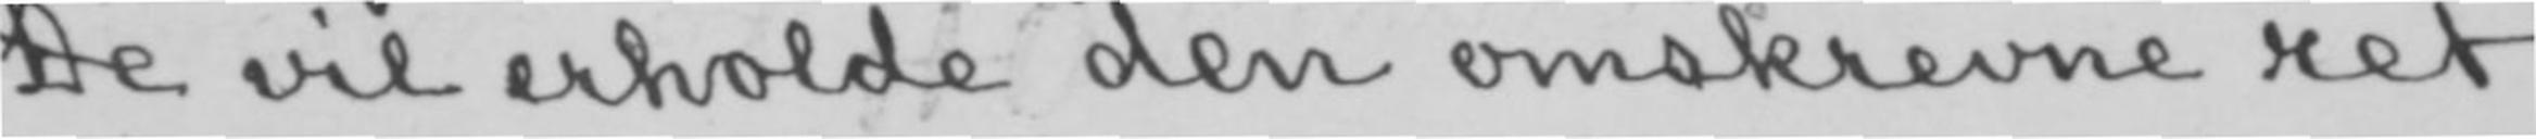De vil erholde den omskrevne ret
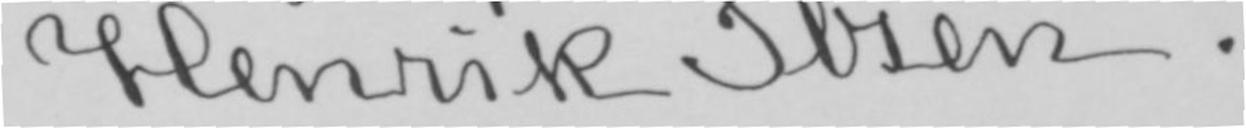Henrik Ibsen.
